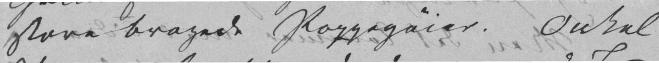store brogede Pappegøier. Onkel
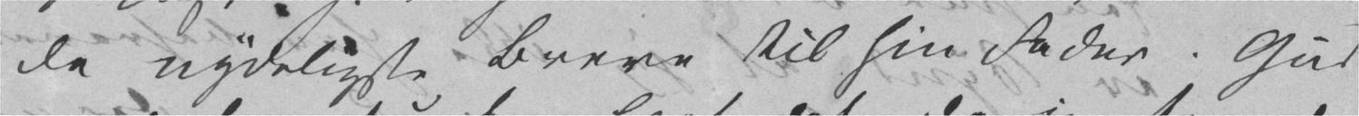de nydeligste Breve til sin Fader. Gud
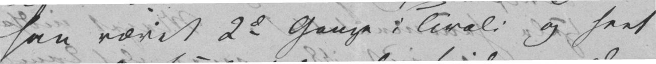han været tvende Gange i Tivoli og seet
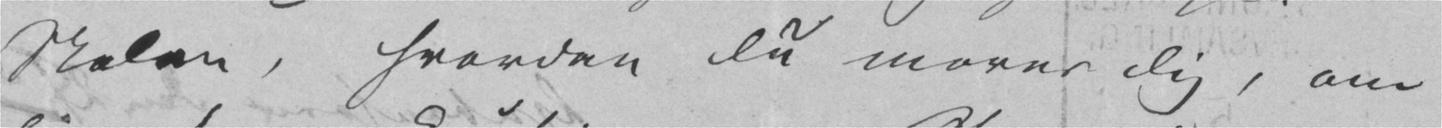Skolen, hvordan Du morer Dig, om
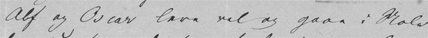Alf og Oscar leve vel og gaae i Skole
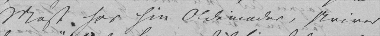Moss hos sin Oldemoder, skriver
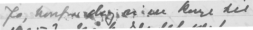Ja, hvorfor drog vi en konge til
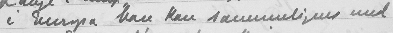i Europa bare kan sammlignes med
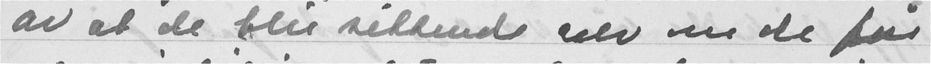av at de blir sittende selv om de får
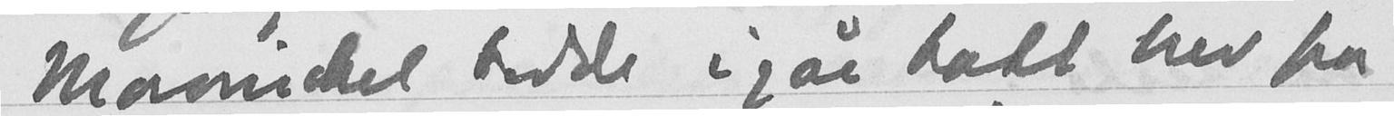Mowinckel hadde i går fått brev fra
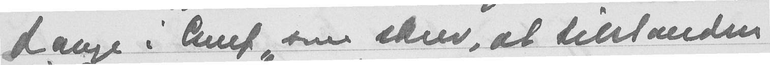Lange i Genf som skrev, at tilstanden
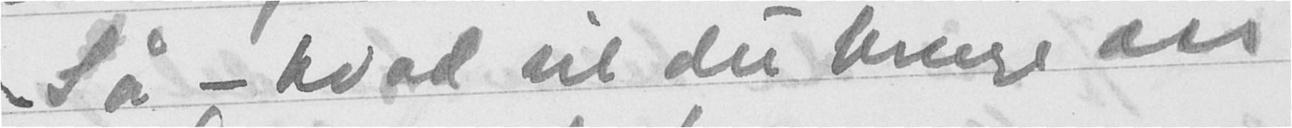Så - hvad vil du bringe oss
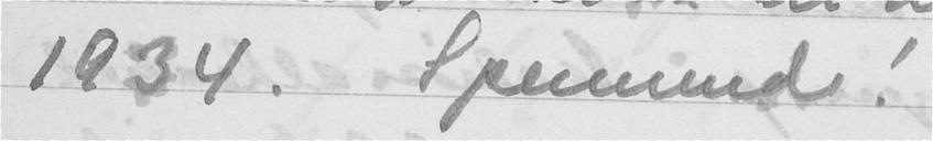1934. Spennende.
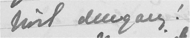hørt dengang!
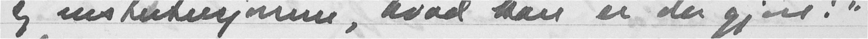sig mindretallsregjering hvad har vi da gjort?"
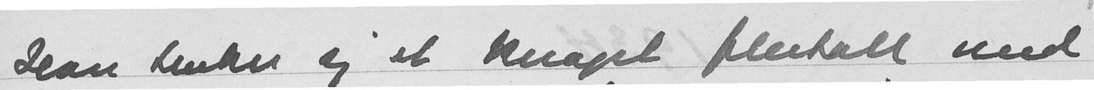Han tenker sig et knapt flertall med
